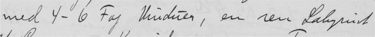med 4-6 Fag Vinduer, en ren Labyrint
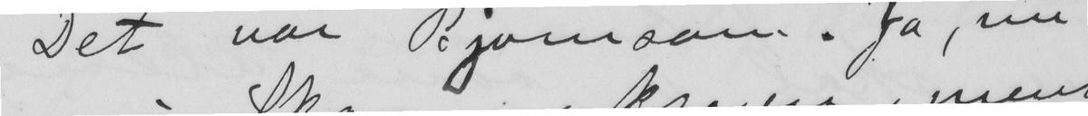Det var Bjørnson. Ja, nu
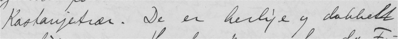Kastanjetrær. De er herlige og dobbelt
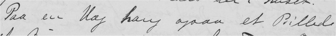Paa en Væg hang ogsaa et Billede
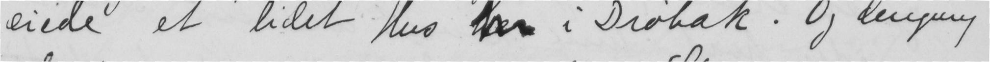eiede et lidet Hus  i Drøbak. Og dengang
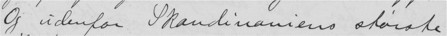Og udenfor Skandinaviens største
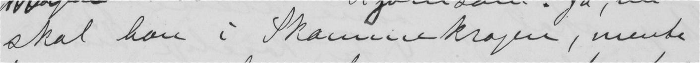skal han i Skammekrogen, mente
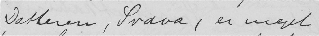Datteren, Svava, er meget
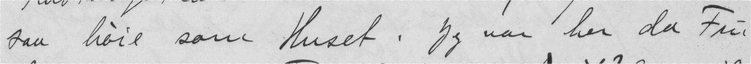saa høie som Huset. Jeg var her da Fru
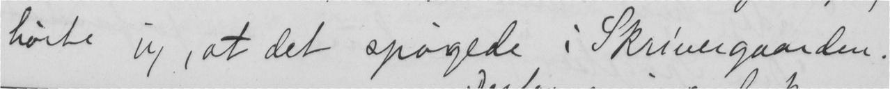hørte jeg, at det spøgede i Skrivergaarden.
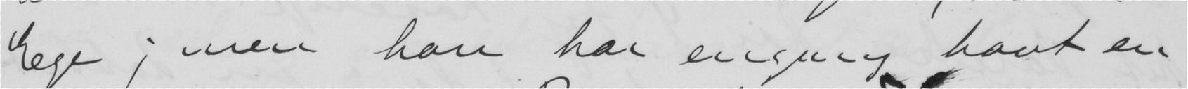Ege; men han har engang havt en
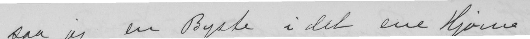saa jeg en Byste i det ene Hjørne
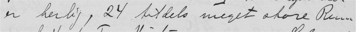er herlig, 24 tildels meget store Rum
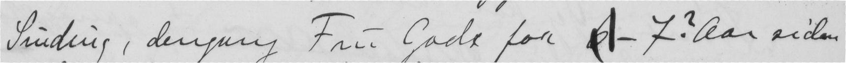Sinding, dengang Fru Gade for  7? Aar siden
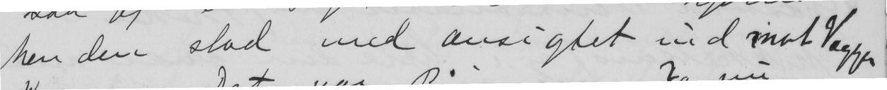Men den stod med ansigtet ind mot Veggen
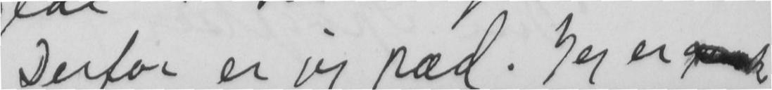Derfor er jeg ræd. Jeg er
